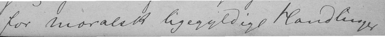for moralsk ligegyldige Handlinger
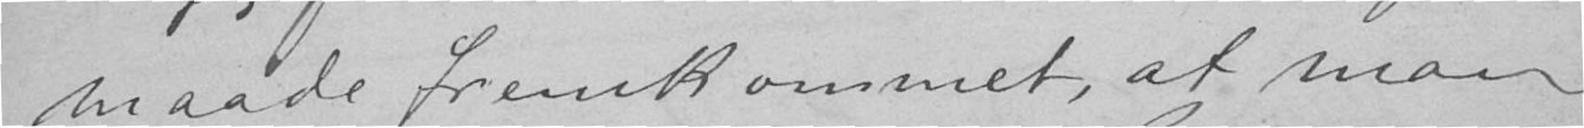maade fremkommet, at man
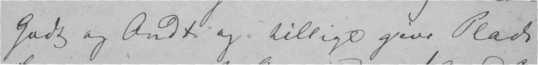Godt og Ondt og tillige give Plads
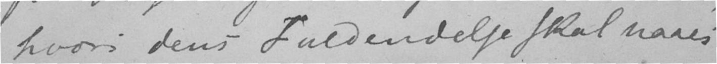hvori dens Fuldendelse skal naaes
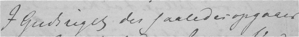I Gudsriget der saaledes opgaaer
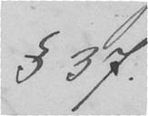§ 37.
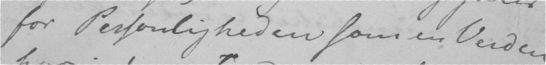for Personligheden som en Verden
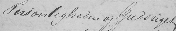Personligheden og Gudsriget
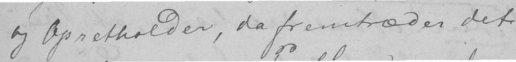og Opretholder, da fremtræder det
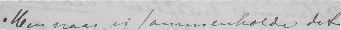Men naar vi sammenholde det
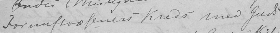Fornuftvæseners Kreds med Guds
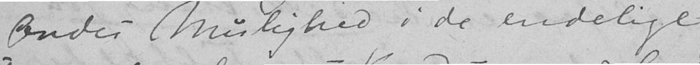Ondes Mulighed i de endelige
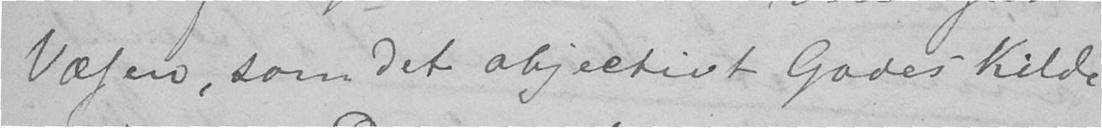Væsen, som det objectivt Godes kilde
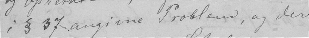i § 37 angivne Problem, og der
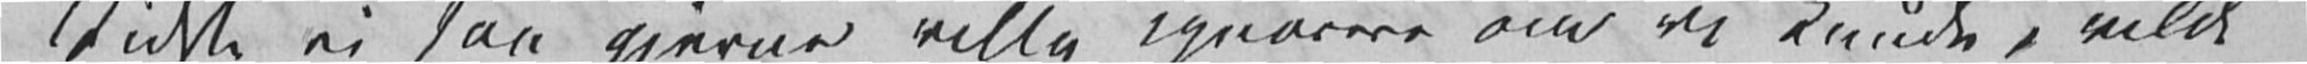Sidste vi saa gjerne ville ignorere om vi kunde, vilde
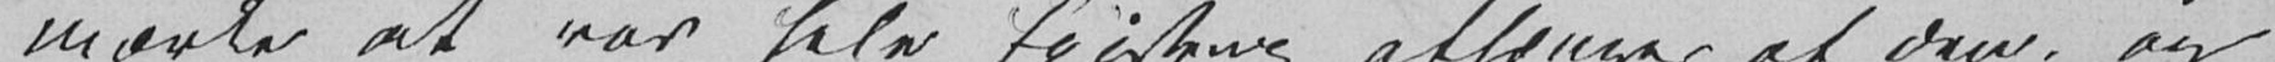mærke at vor hele Existenz afhænger af den, og
